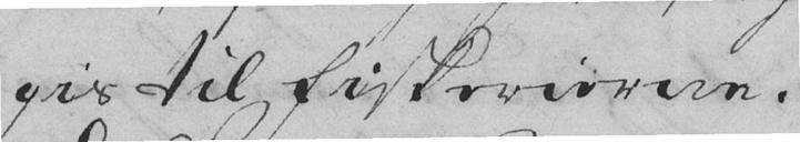gis til Fiskerierne.
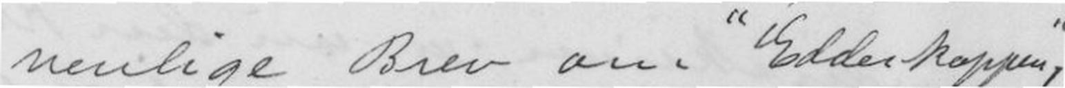venlige Brev om "Edderkoppen",
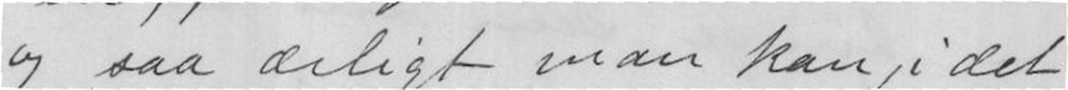og saa ærligt man kan, i det
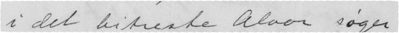i det bitreste Alvor søger
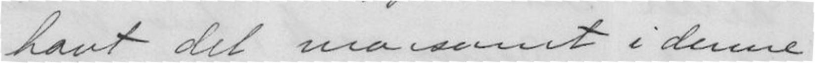havt det morsomt i denne
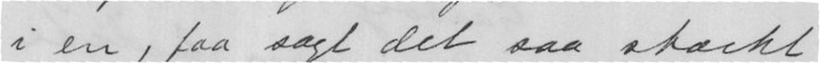i en, faa sagt det saa stærkt
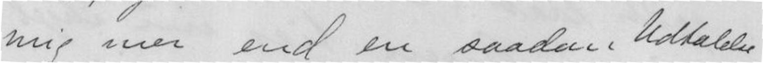mig mer end en saadan Udtalelse
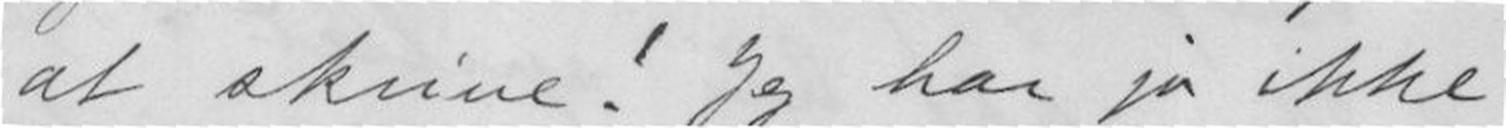at skrive! Jeg har jo ikke
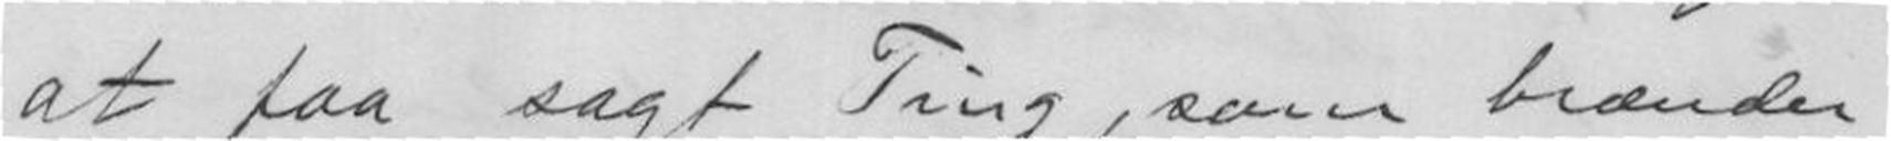at faa sagt Ting, som brænder
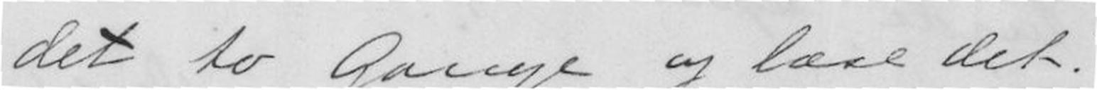det to Gange og læse det!
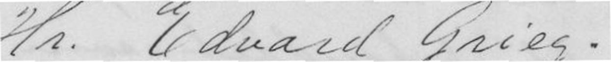Hr. Edvard Grieg!
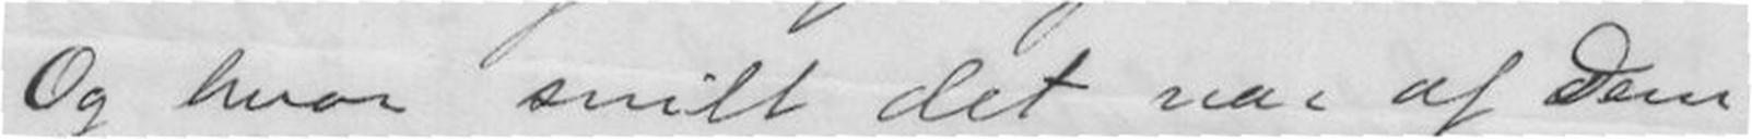Og hvor snilt det var af Dem
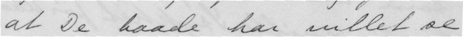at De baade har villet se
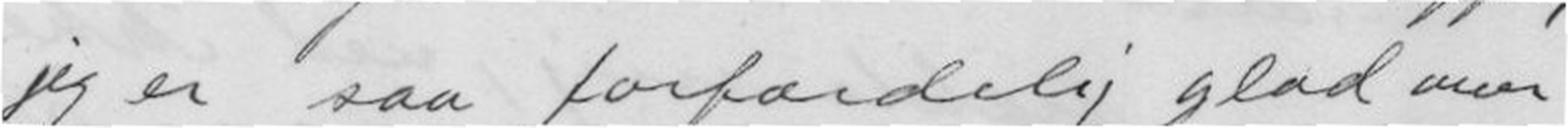jeg er saa forfærdelig glad over
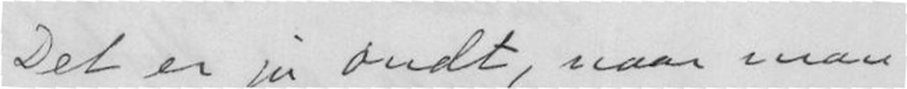Det er jo ondt, naar man
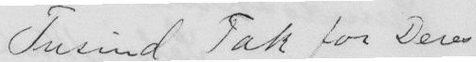Tusind Tak for Deres
